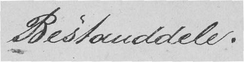Bestanddele.
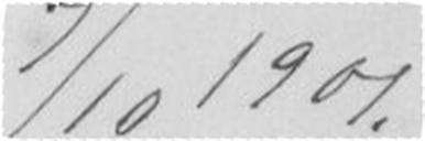7/10 1901
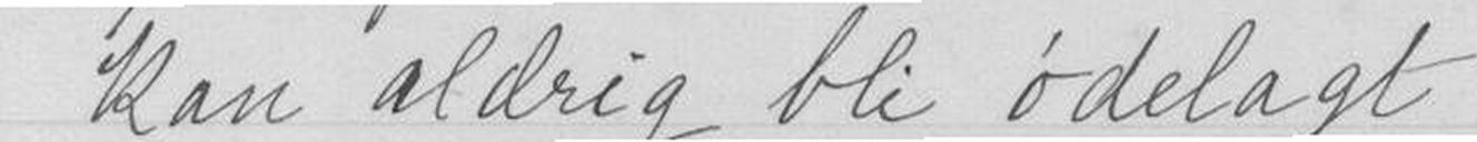kan aldrig bli ødelagt
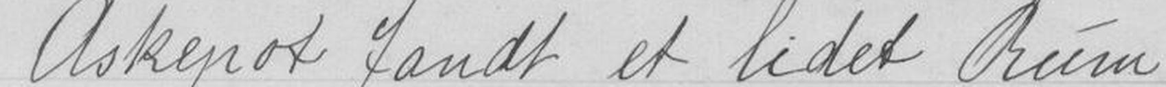Askepot fandt et lidet Rum
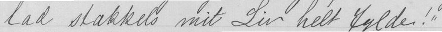lad stakkels mit Liv helt fylde!"
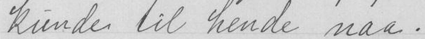kunde til hende naa.
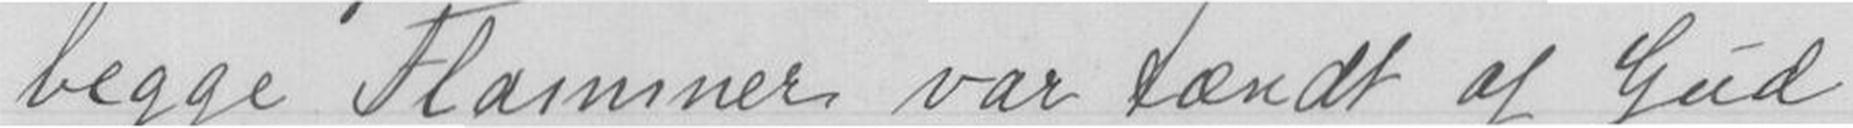begge Flammer var tændt af Gud
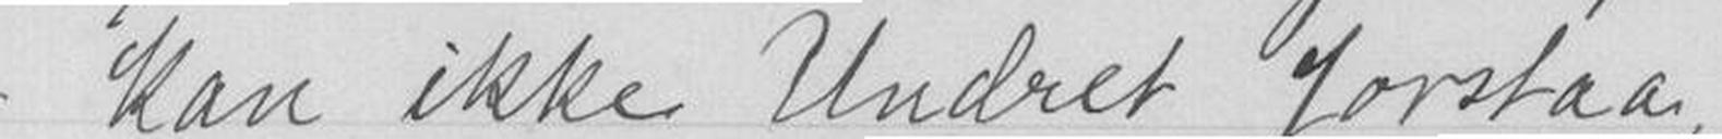- kan ikke Undret forstaa, -
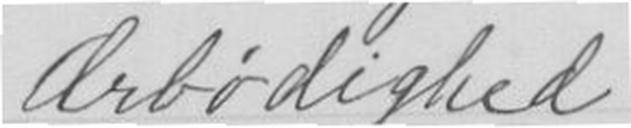Ærbødighed
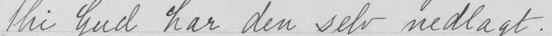thi Gud har den selv nedlagt.
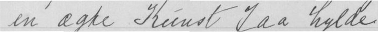en ægte Kunst faa hylde -
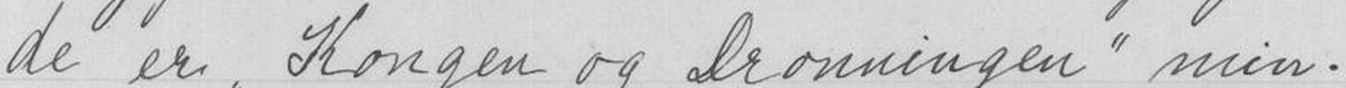de er Kongen og Droningen min.
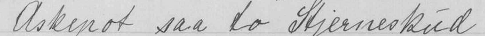Askepot saa to Stjerneskud
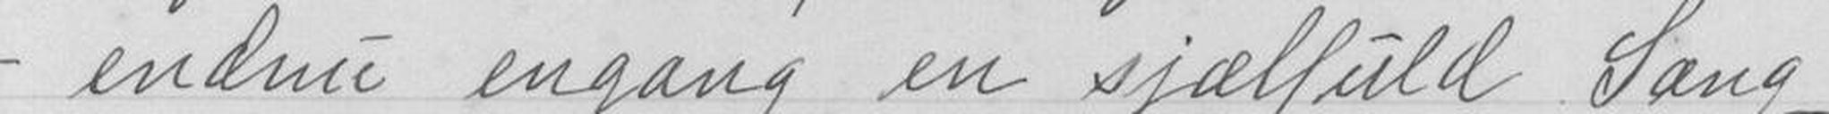- endnu engang en sjælfuld Sang
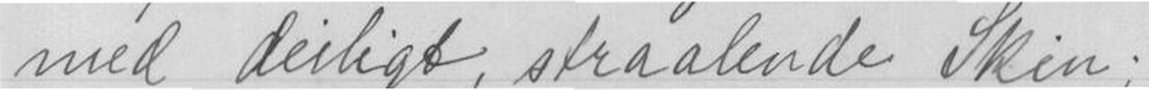med deiligt, straalende Skin,
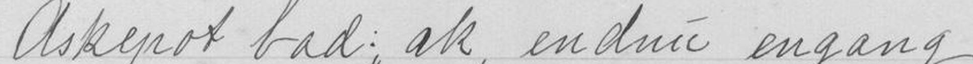Askepot bad; ak, endnu engang
